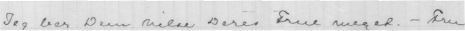Jeg ber Dem hilse Deres Frue meget. - Fru
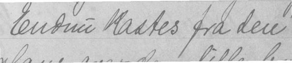Endnu kastes fra den
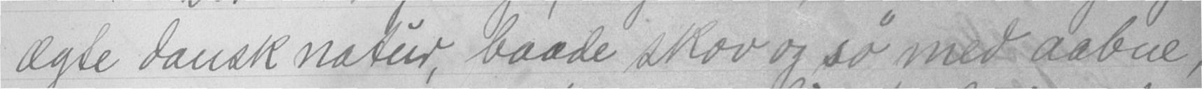ægte dansk natur, baade skov og sø med aabne,
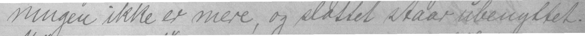ningen ikke er mere, og slottet staar ubenyttet.
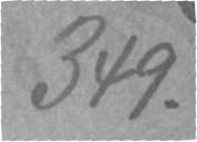349.
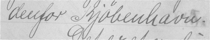denfor Kjøbenhavn.
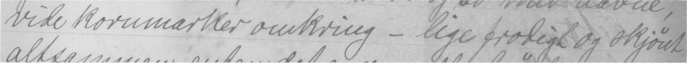vide kornmarker omkring - lige frodigt og skjønt
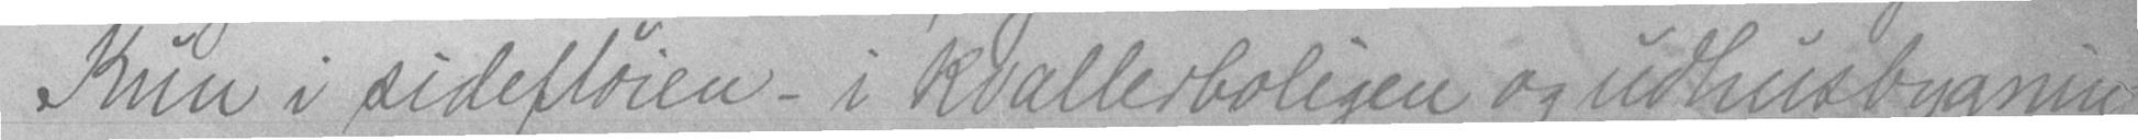Kun i sidefløien - i kvallerboligen og udhusbygnin
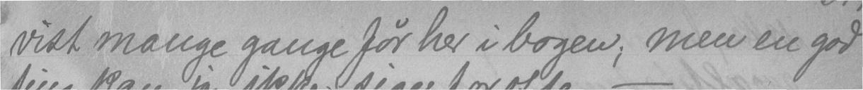vist mange gange før her i bogen; men en god
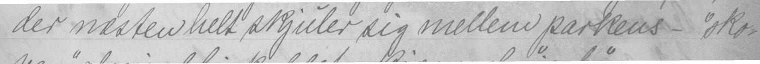der næsten helt skjuler sig mellem parkens - "sko-
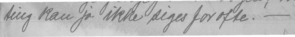ting kan jo ikke siges for ofte. -
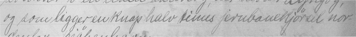og som ligger en knap halv times jernbanekjørsel nor-
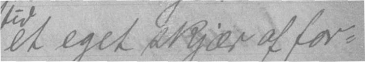et eget skjær af for-
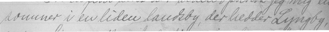sommer i en liden landsby, der hedder Lyngby,
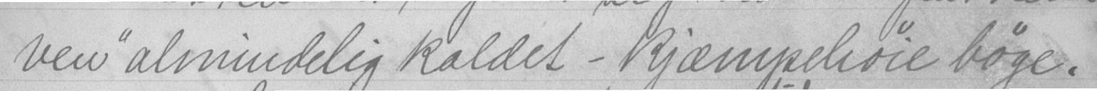ven" almindelig kaldet - kjæmpehøie bøge.
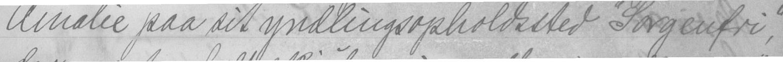Amalie paa sit yndlingsopholdssted "Sorgenfri",
